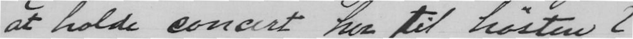at holde concert her til høsten?
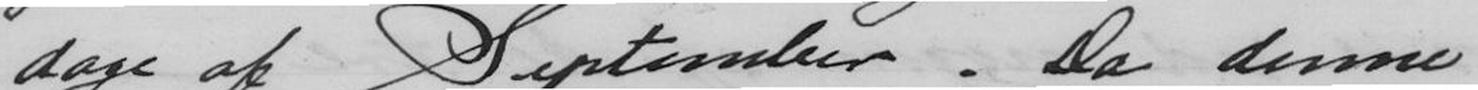dage af September. Da denne
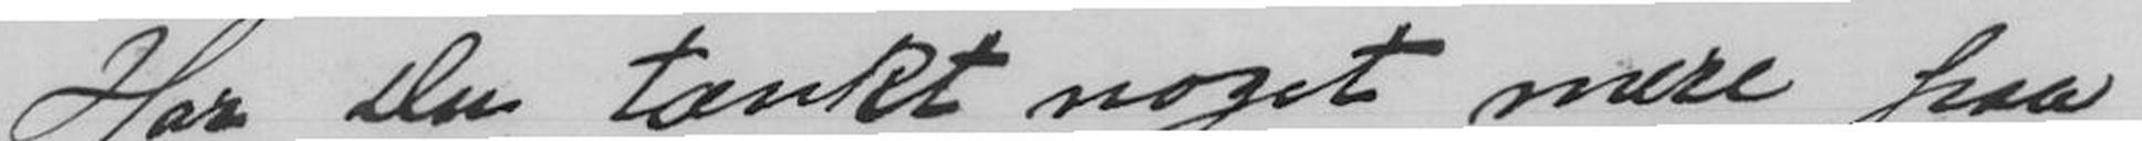Har Du tænkt noget mere paa
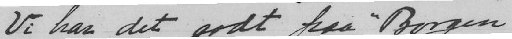Vi har det godt paa "Borgen
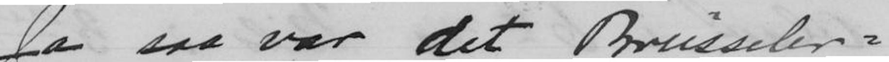Ja saa var det Brüsseler-
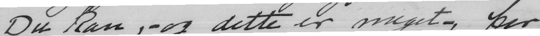Du kan, - og dette er meget -, for
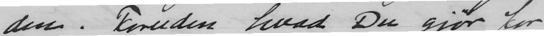den. Foruden hvad Du gjør for
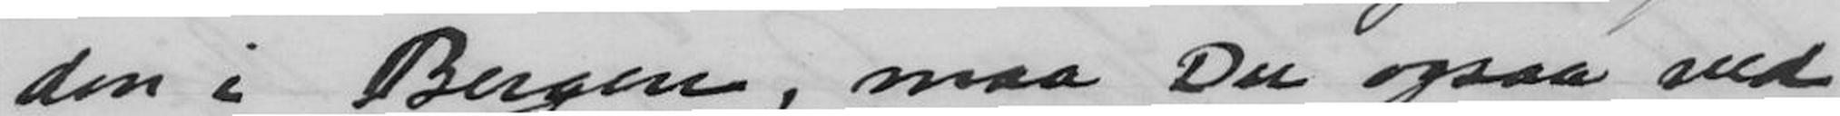den i Bergen, maa Du ogsaa ved
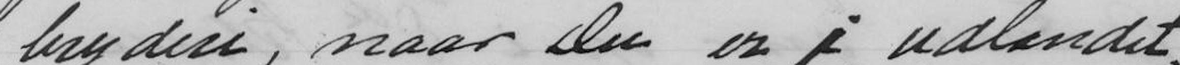bryderi, naar Du er i udlandet.
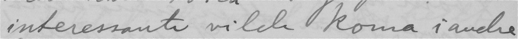interessante vilde koma i andre
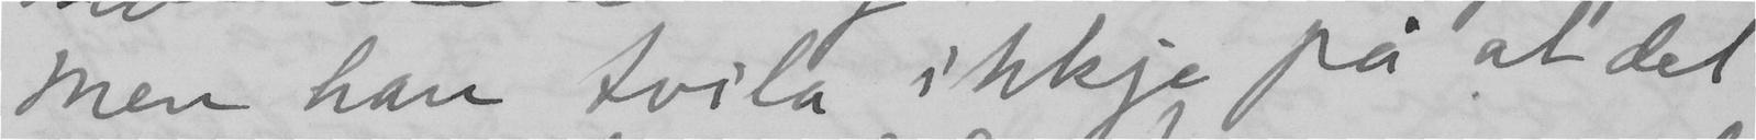Men han tvila ikkje på at det
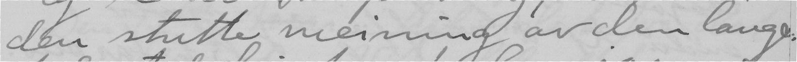den stutte meining av den lange
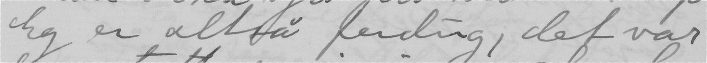Eg er altså ferdug, det var
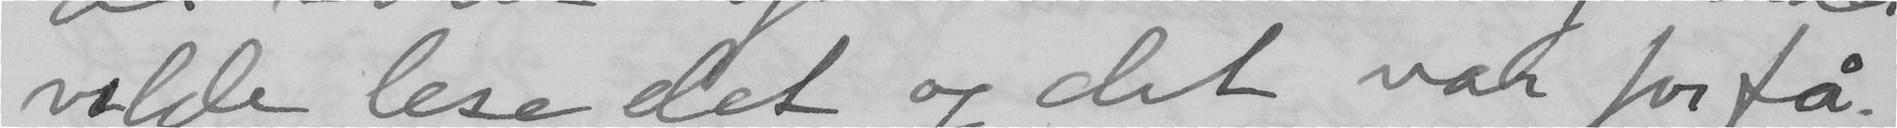vilde lese det og det var for få.
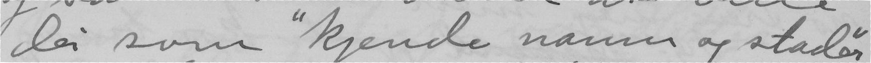dei som "kjende namner og stader"
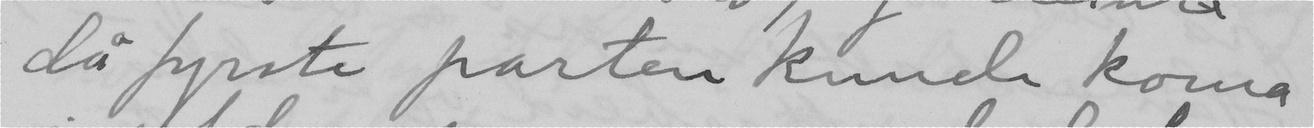då fyrste parten kunde koma
